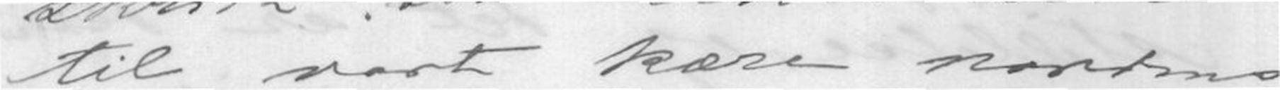til vort kære Nordens
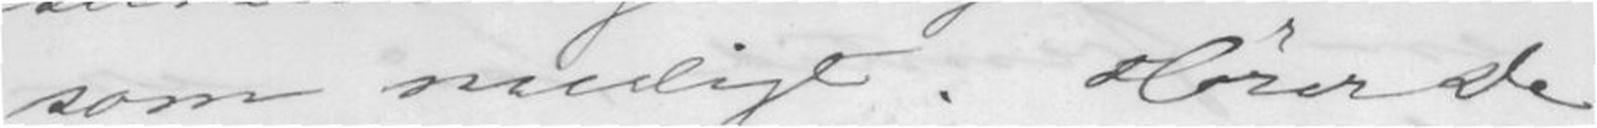som muligt. Hører De.
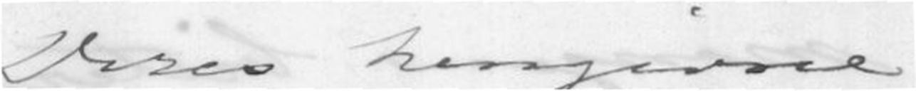Deres hengivne
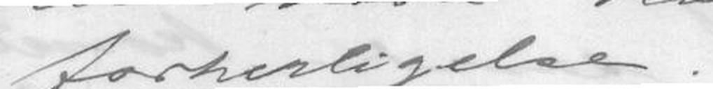forherligelse.
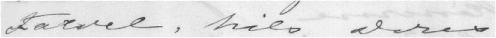Farvel, hils Deres
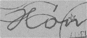Høn
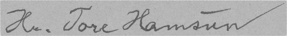Hr. Tore Hamsun
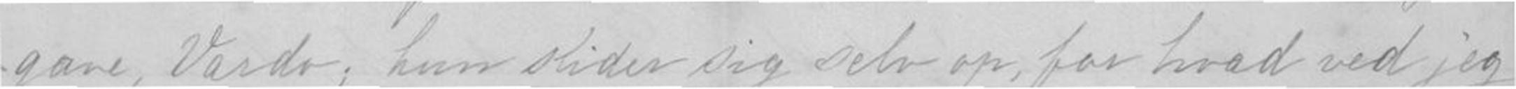gave, Vardo, hun slider sig selv op, for hvad ved jeg
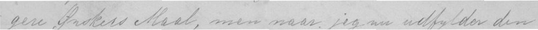gere Ønskers Maal, men naar jeg nu udfylder den
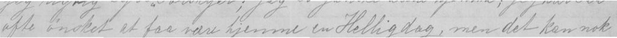ofte ønsket at faa være hjemme en Helligdag, men det kan nok
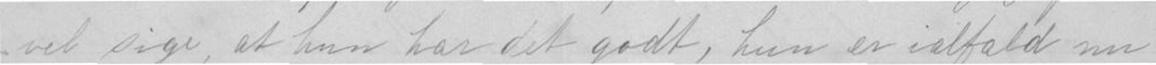vil sige, at hun har det godt, hun er ialfald nu
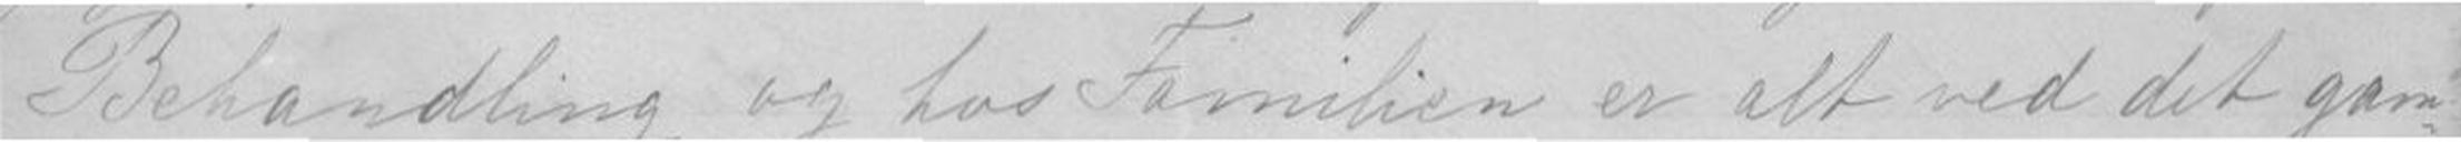Behandling og hos Familien er alt ved det gam-
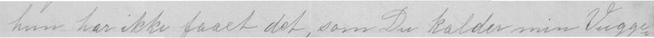hun har ikke faaet det, som Du kalder min Vugge-
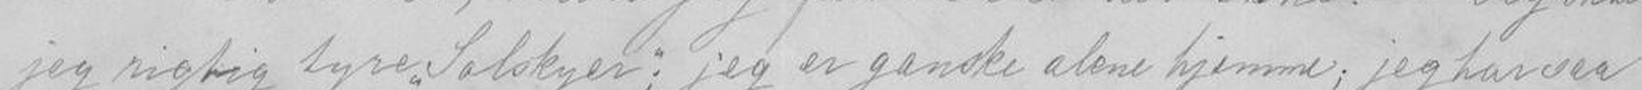jeg rigtig lyre "Solskyer"; jeg er ganske alene hjemme; jeg har saa
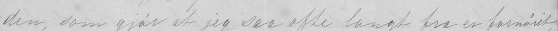den, som gjør at jeg saa ofte langt fra er fornøiet,
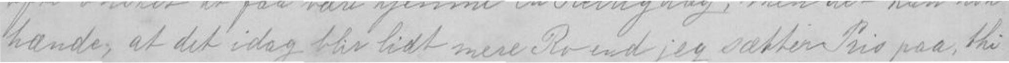hænde, at det idag blir lidt mere Ro end jeg sætter Pris paa, thi
